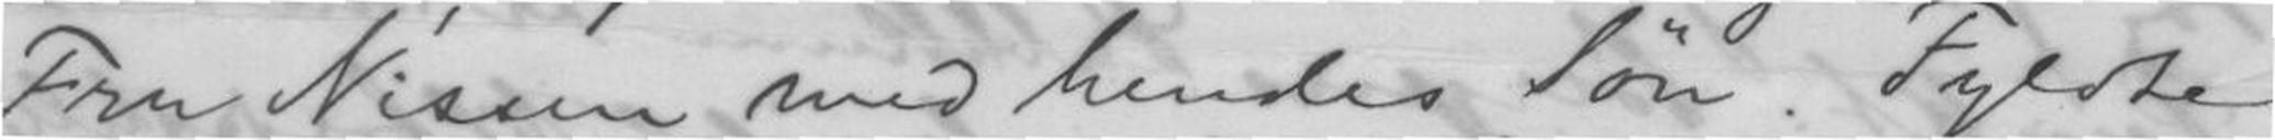Fru Nissen med hendes Søn. Fyldte
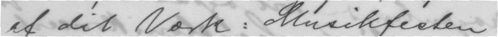af dit Værk: Musikfesten. -
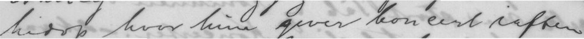herop hvor hun giver Concert iaften
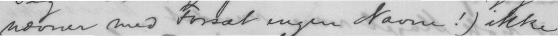nævner med Forsæt ingen Navne!) ikke
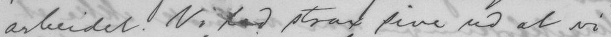arbeidet. Vi lod strax sive ud at vi
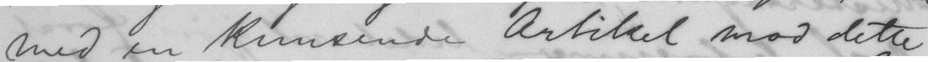med en knusende Artikel mod dette
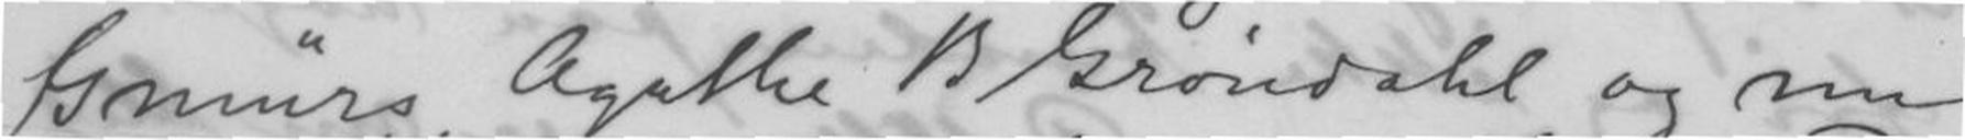Gmürs, Agathe B Grøndahl og nu
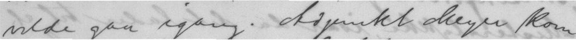vilde gaa igang. Adjunkt Meyer kom
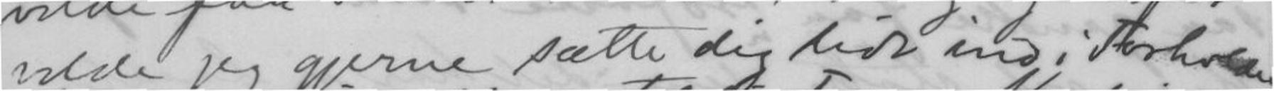vilde jeg gjerne sætte dig lidt ind i Forholdene
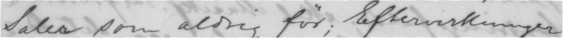Saler, som aldrig før; Eftervirkninger
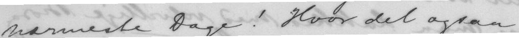nærmeste Dage! Hvor det ogsaa
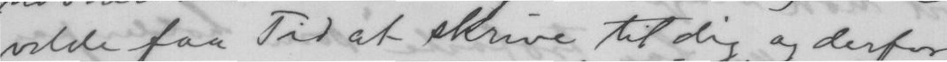vilde faa Tid at skrive til dig og derfor
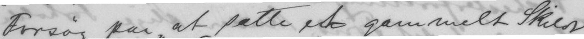Forsøg paa at sætte et gammelt Skildt
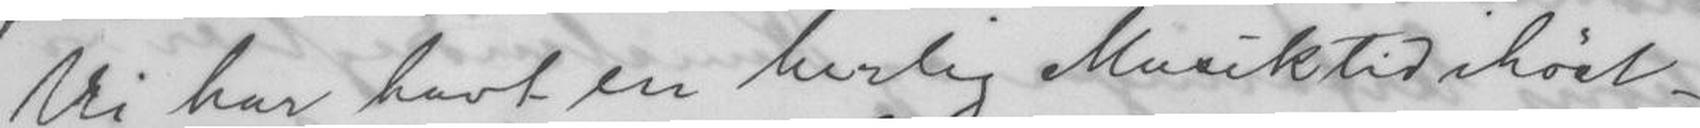Vi har havt en herlig Musiktid ihøst -
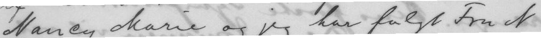Nancy Marie og jeg har fulgt Fru N
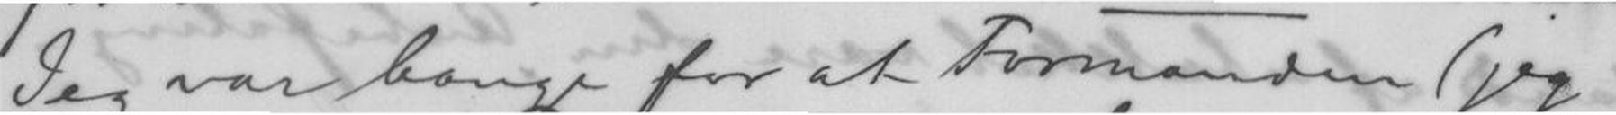Jeg var bange for at Formanden (jeg
